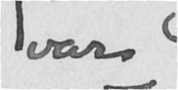var
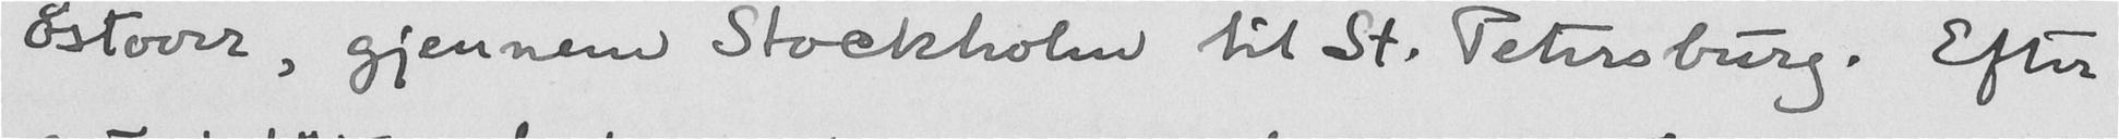østover, gjennem Stockholm til St. Petersburg. Efter
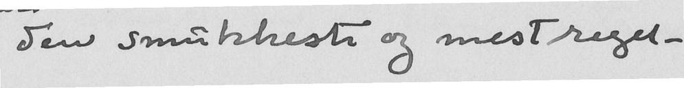den smukkeste og mest regel-
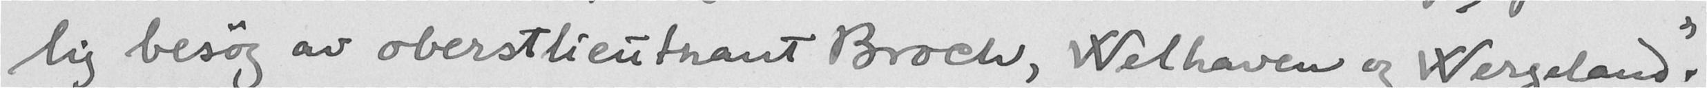lig besøg av oberstlieutnant Broch, Welhaven og Wergeland."
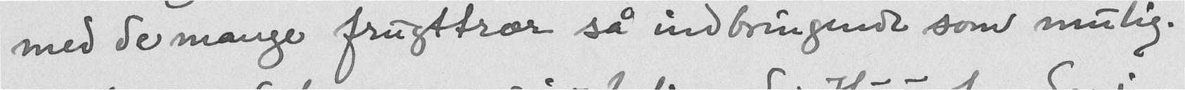med de mange frugttrær så indbringende som mulig.
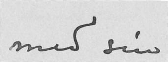med sin
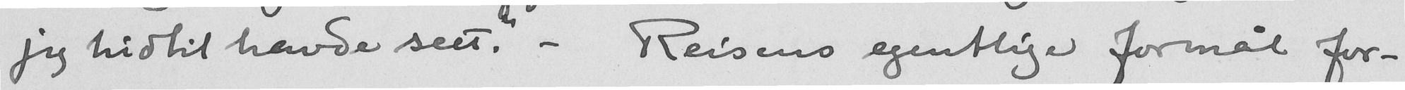jeg hidtil havde seet. - Reisens egentlige formål for-
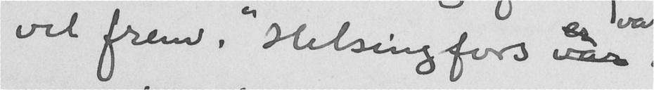vel frem. "Helsingfors var
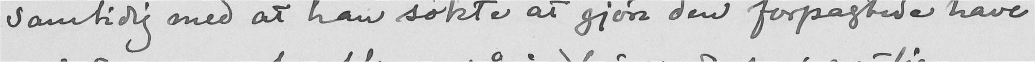samtidig med at han søkte at gjøre den forpagtede have
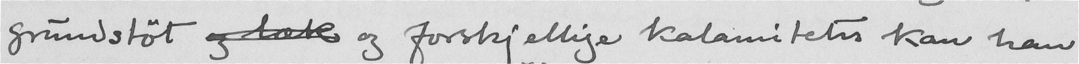grundstøt og læk og forskjellige kalamiteter kom han
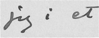jeg i et
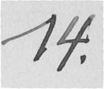14.
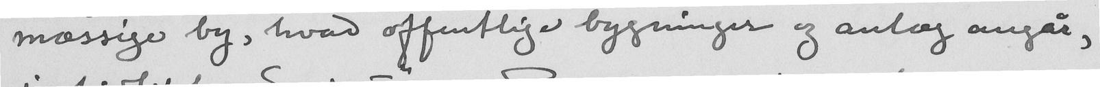mæssige by, hvad offentlige bygninger og anlæg angår,
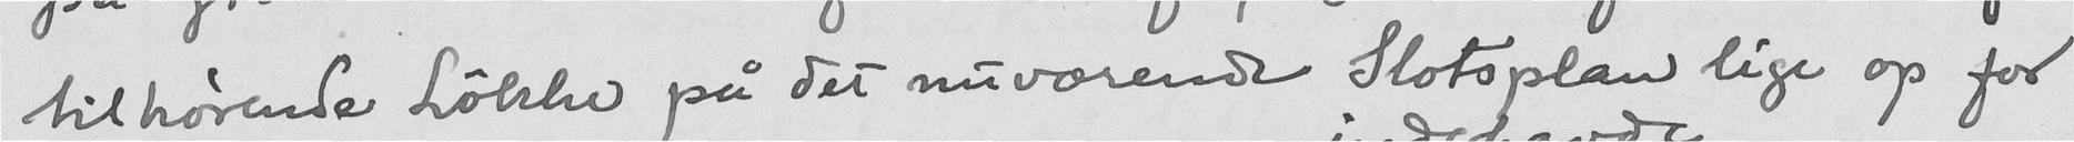tilhørende Løkke på det nuværende Slotsplan lige op for
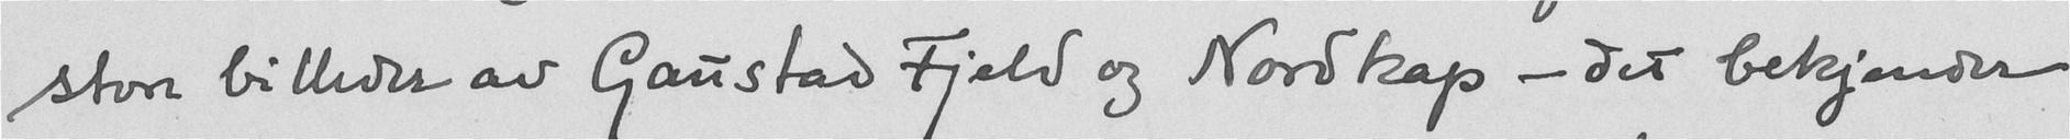store billeder av Gaustad Fjeld og Nordkap - det bekjender
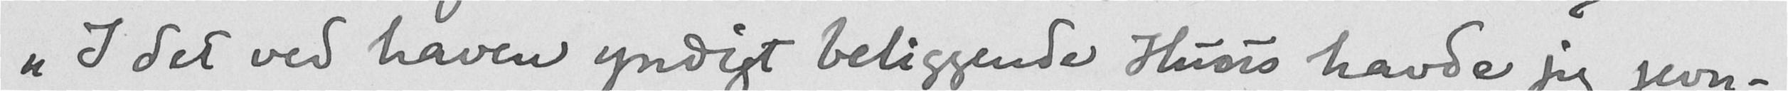"I det ved haven yndigt beliggende Huus havde jeg jevn-
